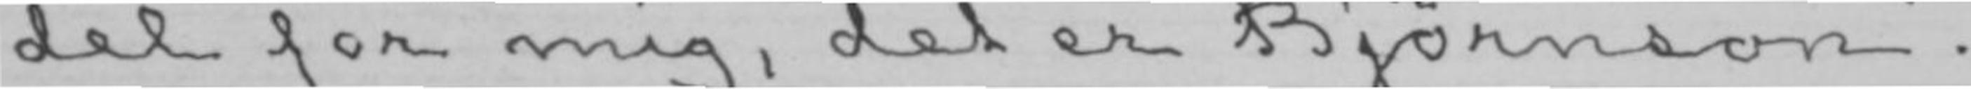del for mig, det er Bjørnson.
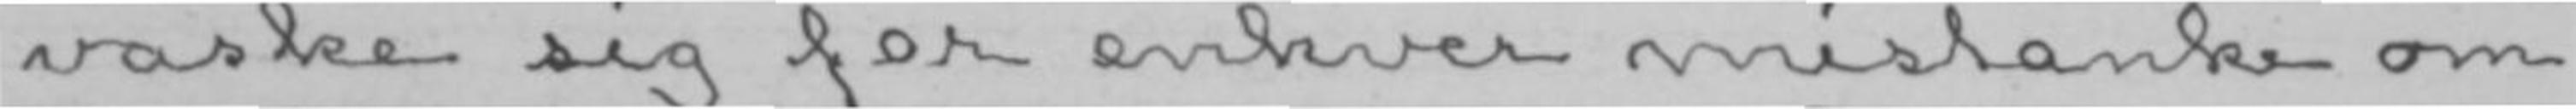vaske sig for enhver mistanke om
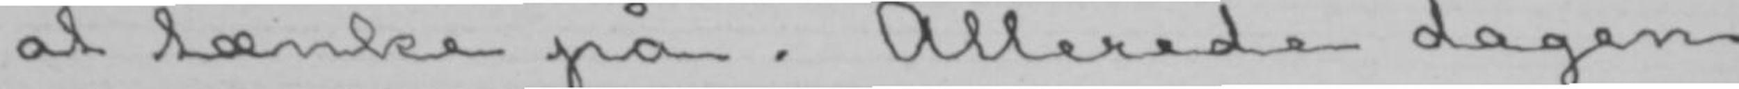at tænke på. Allerede dagen
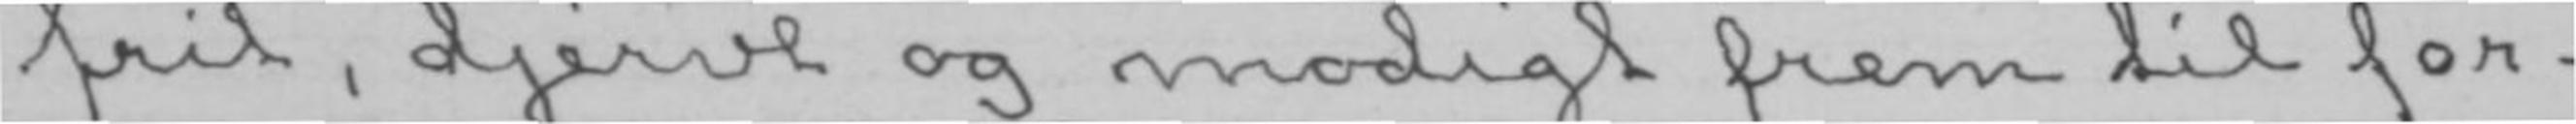frit, djervt og modigt frem til for-
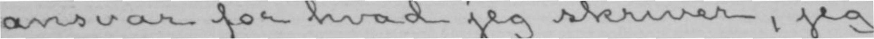ansvar for hvad jeg skriver, jeg
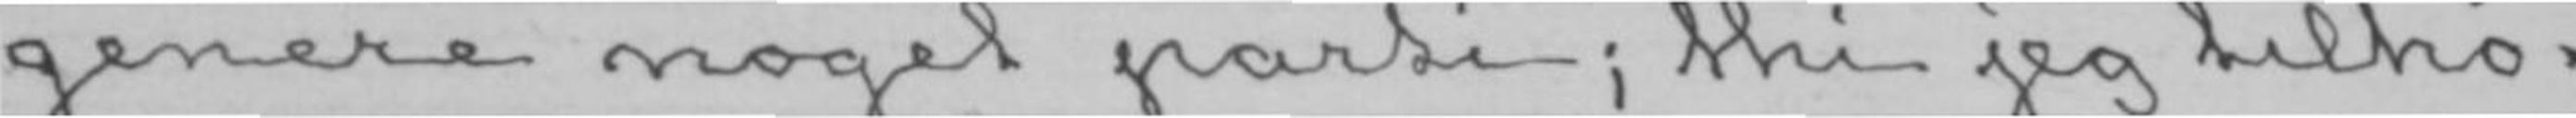genere noget parti; thi jeg tilhø-
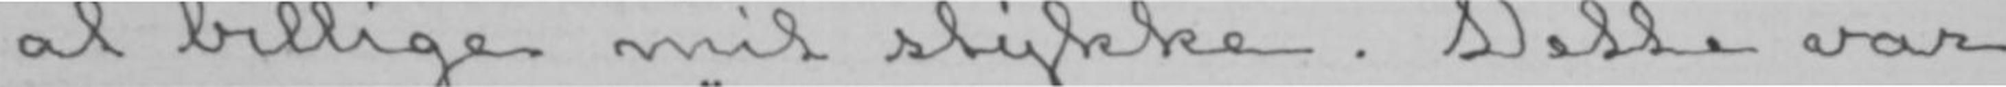at billige mit stykke. Dette var
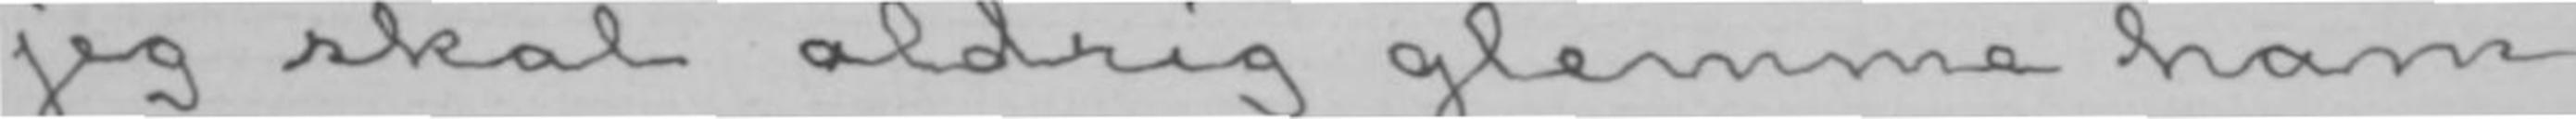jeg skal aldrig glemme ham
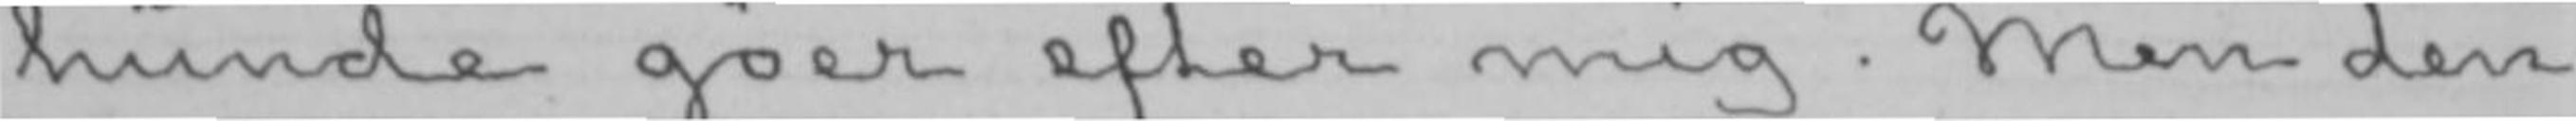hunde gøer efter mig. Men den
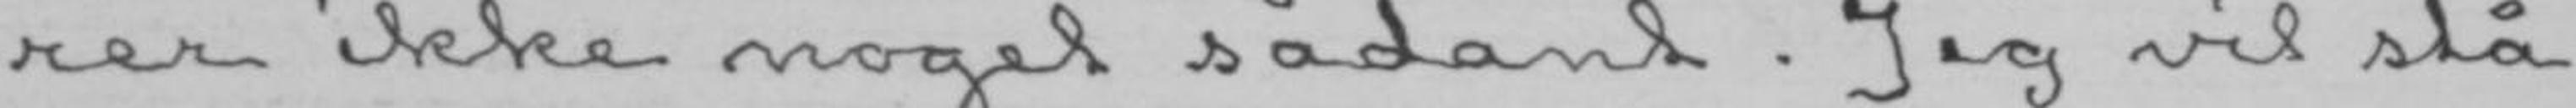rer ikke noget sådant. Jeg vil stå
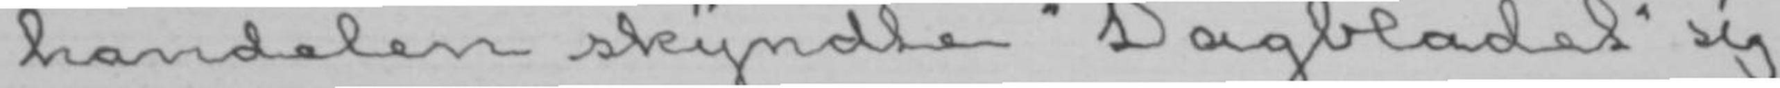handelen skyndte «Dagbladet» sig
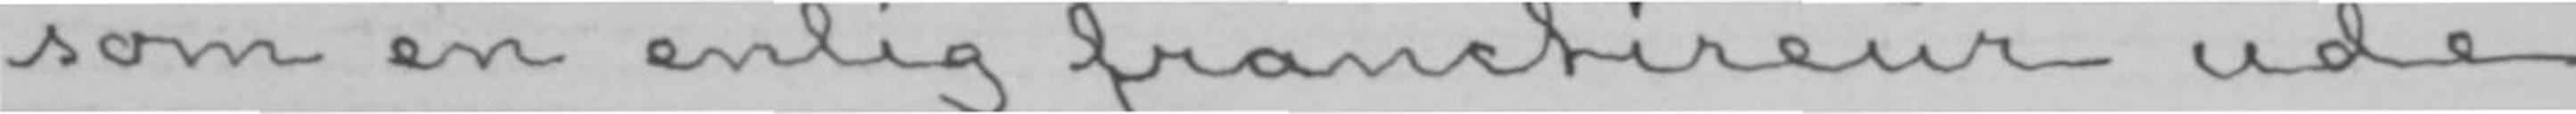som en enlig franctireur ude
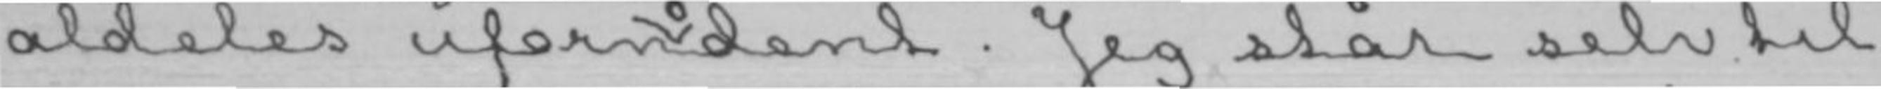aldeles ufornødent. Jeg står selv til
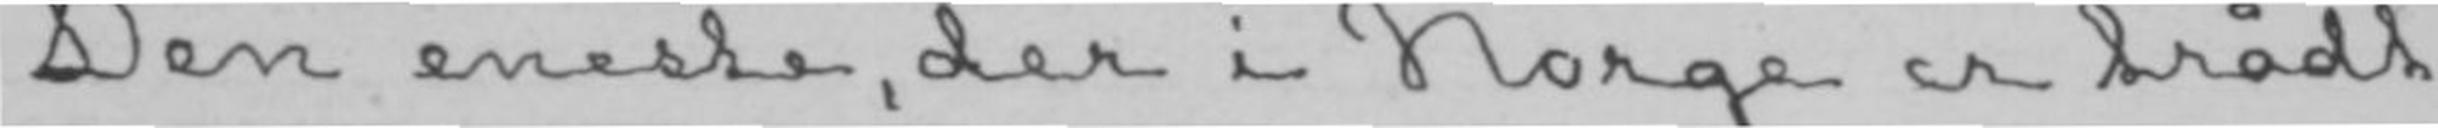Den eneste, der i Norge er trådt
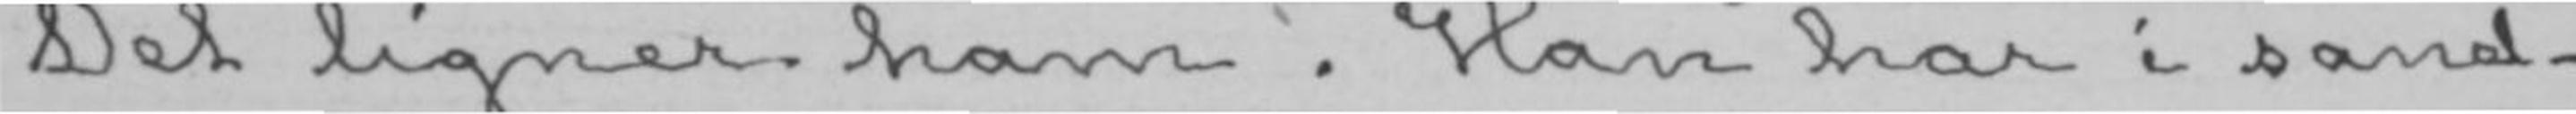Det ligner ham. Han har i sand-
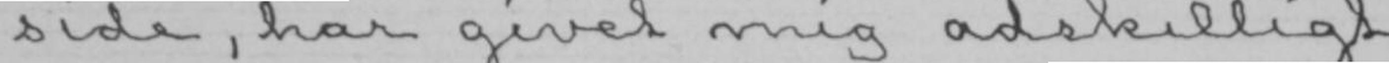side, har givet mig adskilligt
